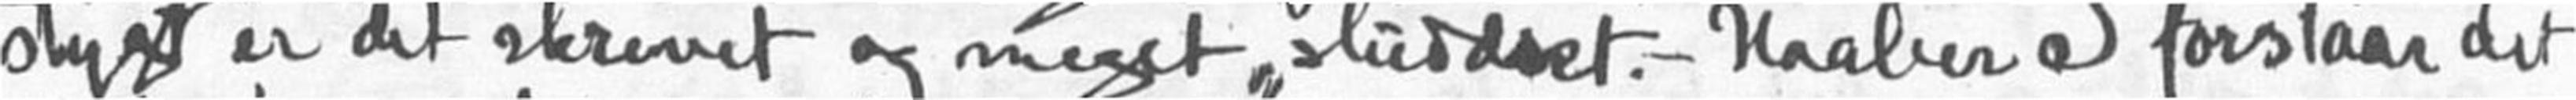stygt er det skrevet og meget "sluddret". - Haaber I forstaar det
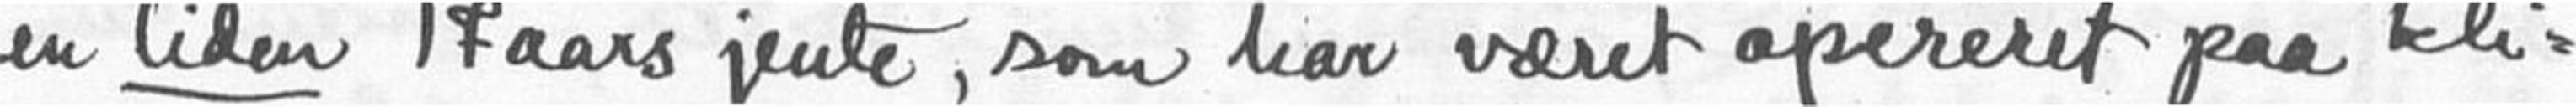en liden 17 aars jente, som har været opereret paa kli-
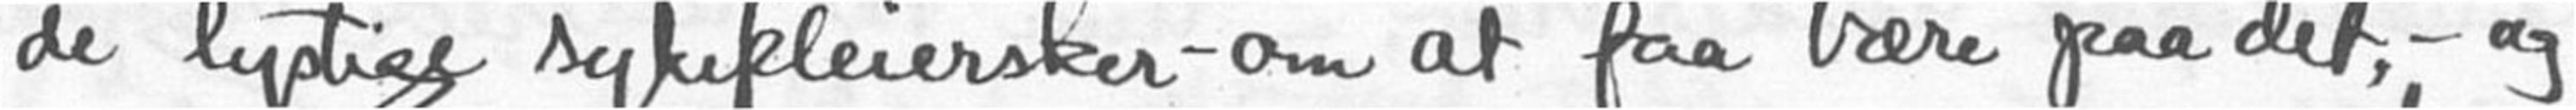de lystige sykepleiersker om at faa bære paa det, - og
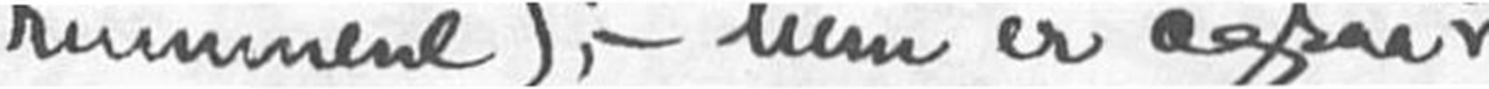rummene); - hun er ogsaa
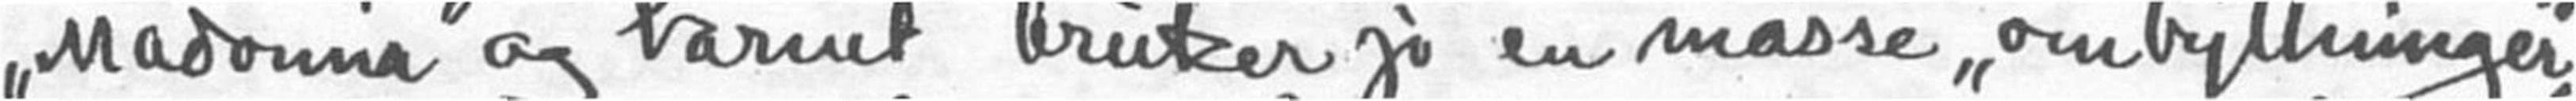"Madonna" og barnet bruker jo en masse "ombyttinger"
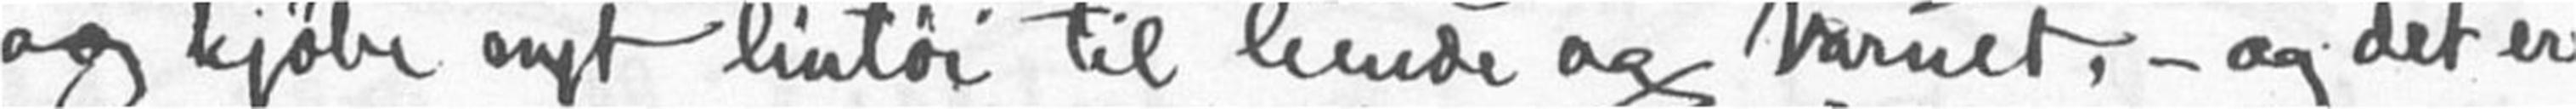og kjøbe nyt lintøi til hende og barnet, - og det er
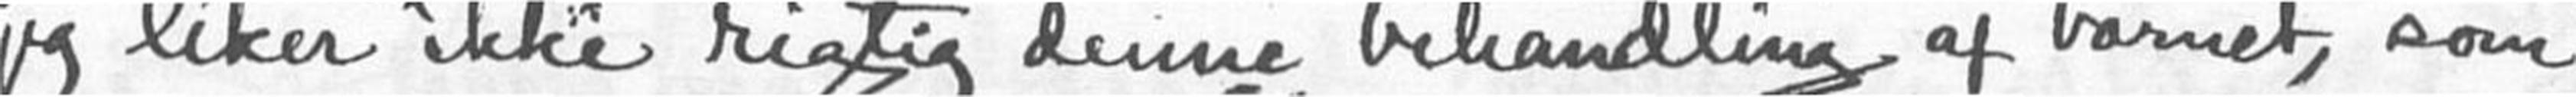jeg liker ikke rigtig denne behandling af barnet, som
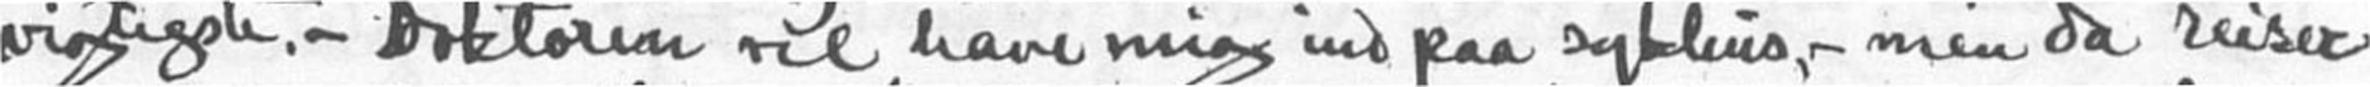vigtigste. - Doktoren vil have mig ind paa sykehus, - men da reiser
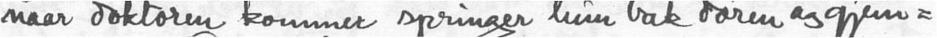naar doktoren kommer springer hun bak døren og gjem-
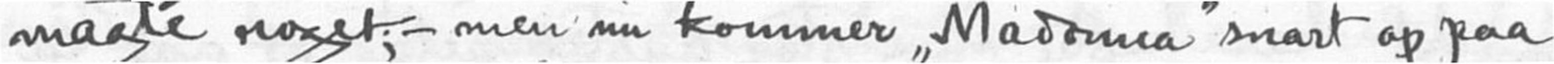magte noget, - men nu kommer "Madonna" snart op paa
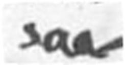saa
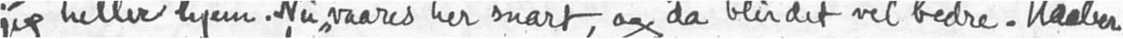jeg heller hjem. Nu "vaares" her snart, og da blir det vel bedre. Haaber
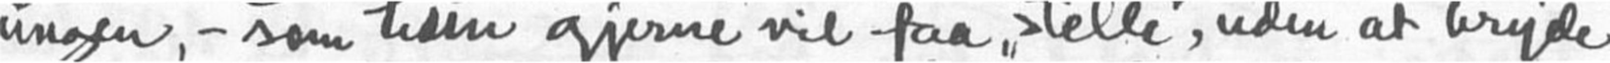ungen, - som hun gjerne vil faa "stelle", uden at bryde
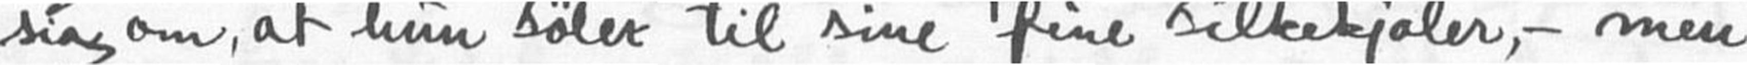sig om at hun søler til sine fine silkekjoler, - men
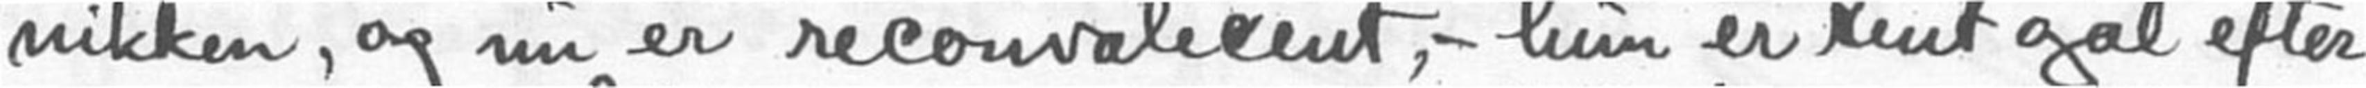nikken, og nu er reconvalecent, - hun er rent gal efter
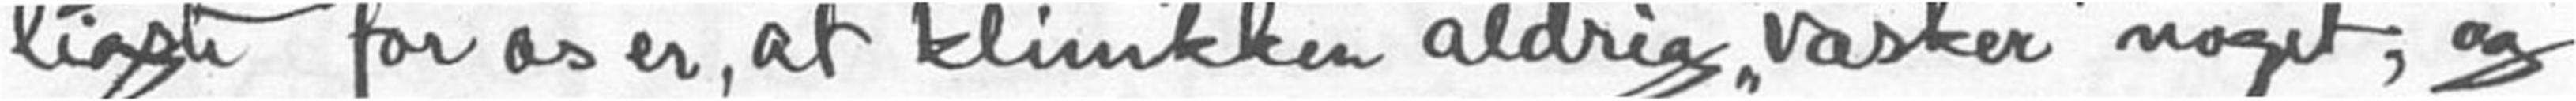ligste for os er, at klinikken aldrig "vasker" noget, og
